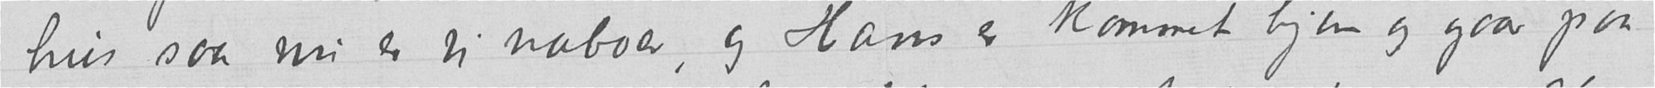hus saa nu er vi naboer, og Hans er kommet hjem og gaar paa
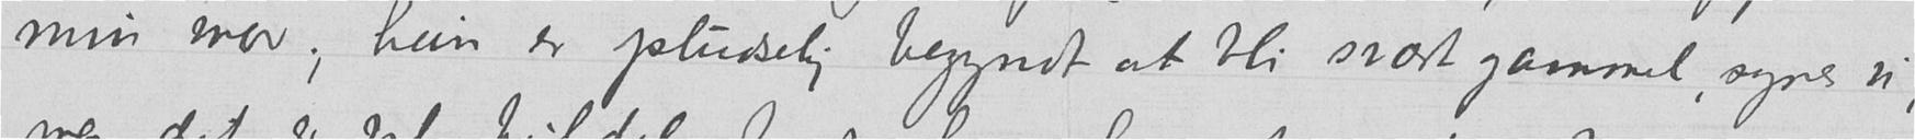min mor; hun er pludselig begyndt at bli svært gammel, synes vi,
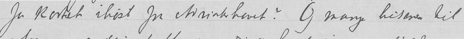for kortet ihøst fra Adriaterhavet? Og mange hilsener til
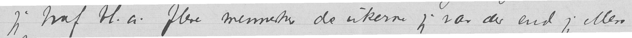jeg traf bl.a. flere mennesker de ukerne jeg var der end jeg ellers
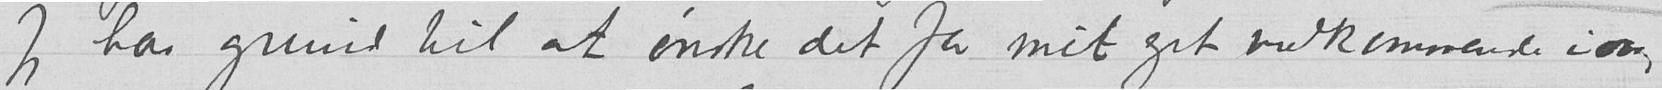– Jeg har grund til at ønske det for mit eget vedkommende iaar,
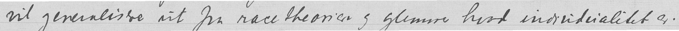vil generalisere ut fra racetheorier og glemmer hvad individualitet er.
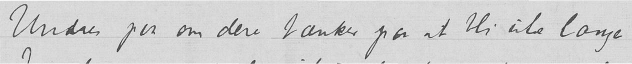Undres paa om dere tænker paa at bli ute længe
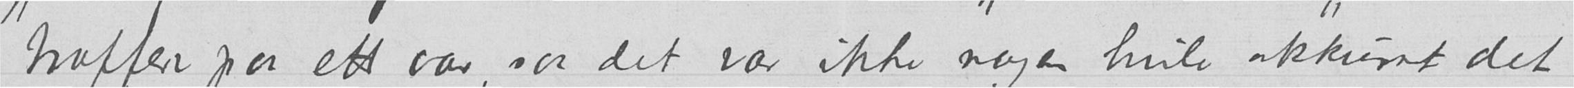træffer paa ett aar, saa det var ikke nogen hvile akkurat det
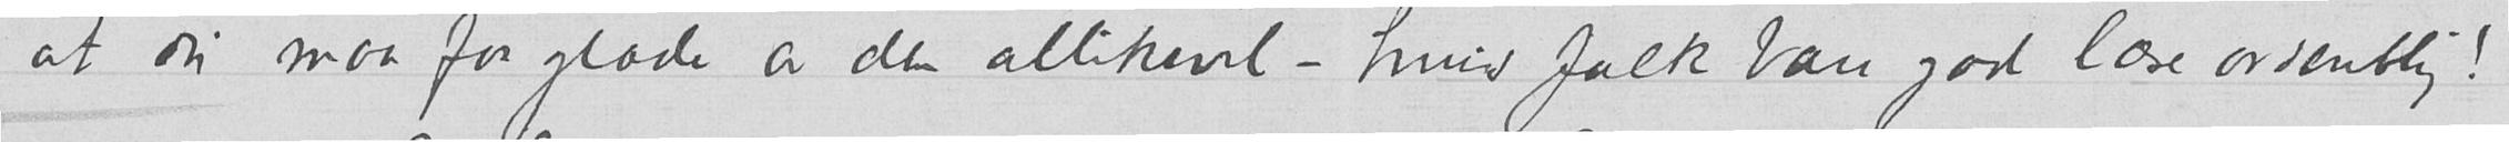at du maa faa glæde av den allikevel – hvis folk bare gad læse ordentlig!
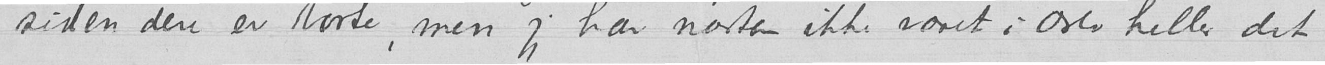siden dere er borte, men jeg har næsten ikke været i Oslo heller det
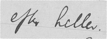efter heller.
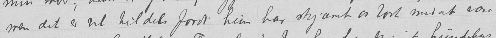men det er vel tildels fordi hun har skjæmt os bort med at være
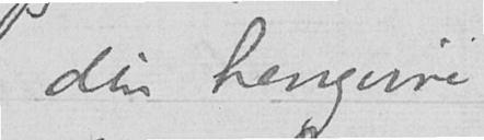din hengivne
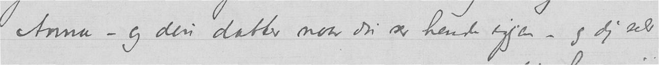Anna – og din datter naar du ser hende igjen – og dig selv
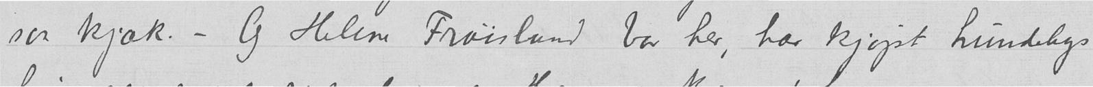saa kjæk. – Og Helene Frøisland bor her, har kjøpt Lundebys
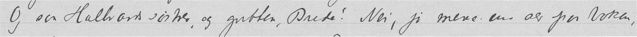Og saa Hallvards søstrer, og gutten, Brede! Nei, jo mere en ser paa boken,
